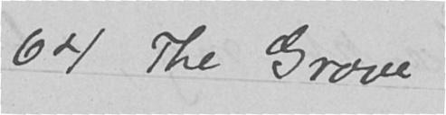64 The Grove
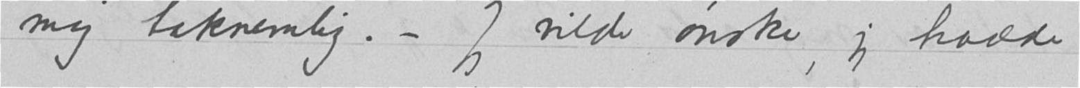mig taknemlig. – Jeg vilde ønske jeg hadde
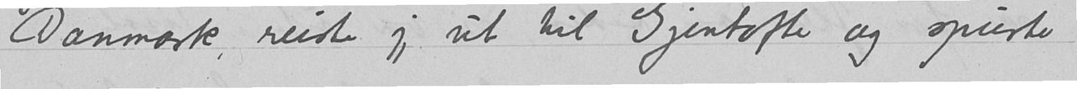Danmark, reiste jeg ut til Gjentofte og spurte
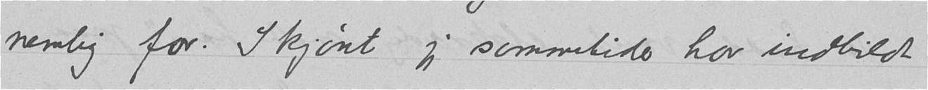nemlig for. Skjønt jeg sommetider har indbildt
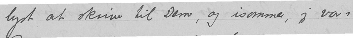lyst at skrive til Dem, og isommer, jeg var i
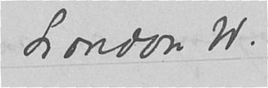London W.
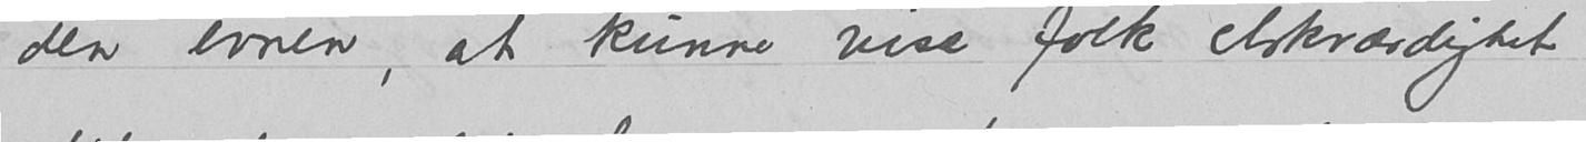den evnen, at kunne vise folk elskværdighet
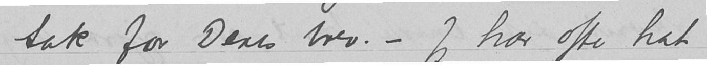tak for Deris brev. – Jeg har ofte hat
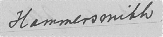Hammersmith
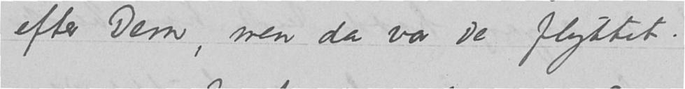efter Dem, men da var de flyttet.
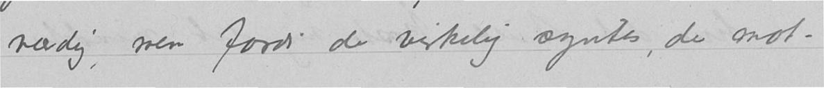værdig, men fordi de virkelig syntes, de mot-
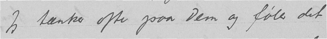Jeg tænker ofte paa Dem og føler det
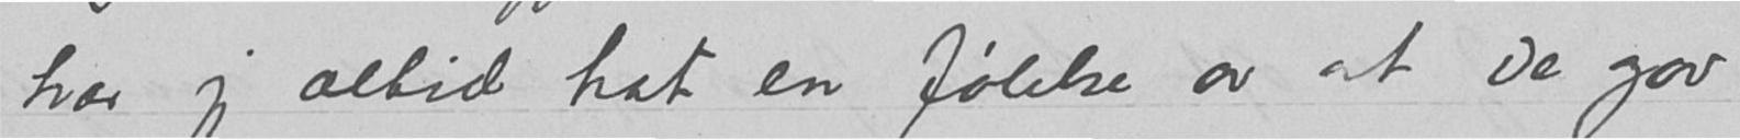har jeg altid hat en følelse av at de gav
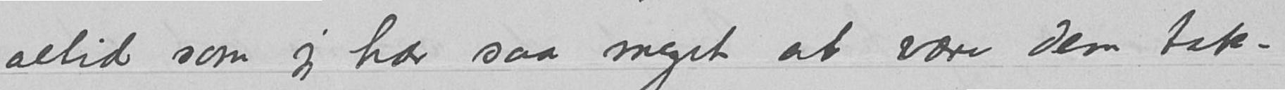altid som jeg har saa meget at være Dem tak-
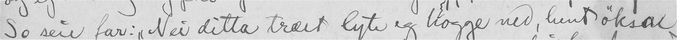So seie far: "Nei ditta træet lyte eg høgge ned, hent øksa
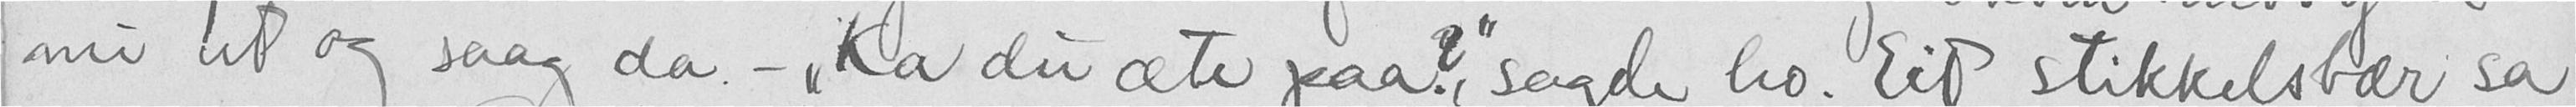mi ut og saag da, - "Ka du æte paa?" sagde ho. Eit stikkelsbær sa
